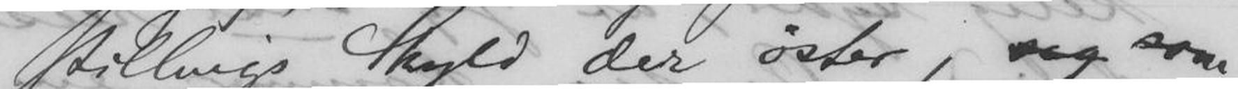stillings Skyld der øster, sig som
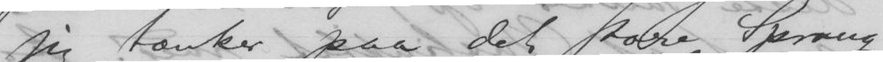jeg tænker paa det store Sprang
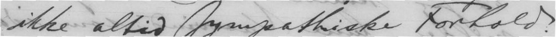ikke altid sympathiske Forhold.
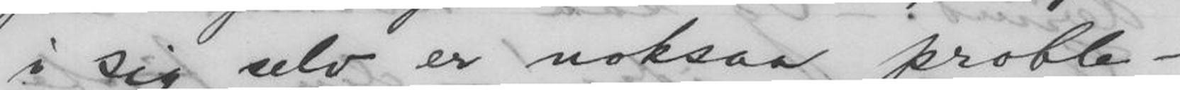i sig selv er noksaa proble-
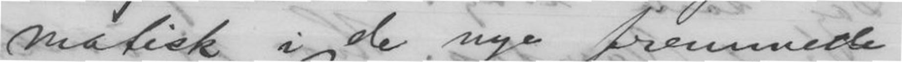matisk i de nye fremmede,
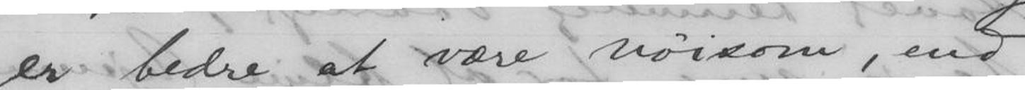er bedre at være nøisom, end
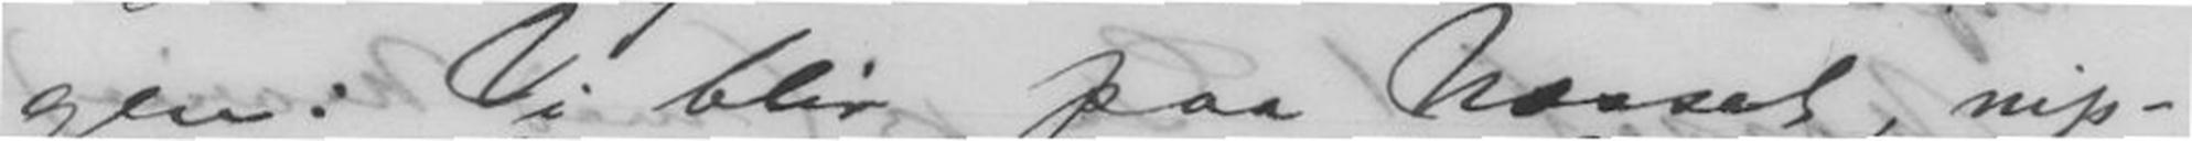gen: Vi blir paa Næsset, nip-
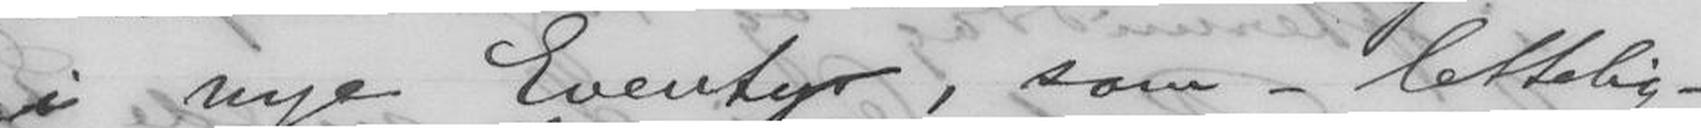i nye Eventyr, som - lettelig -
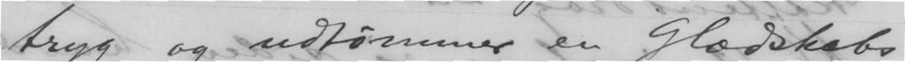tryg og udtømmer en Glædskabs
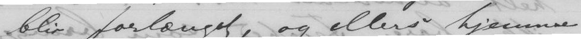blir forlænget, og ellers hjemme
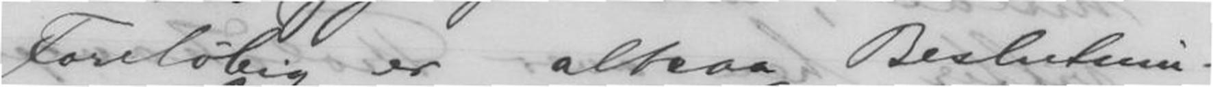Foreløbig er altsaa Beslutnin-
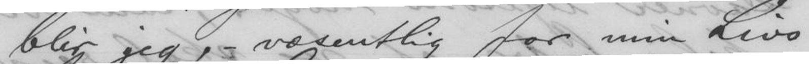blir jeg, - væsentlig for min Livs-
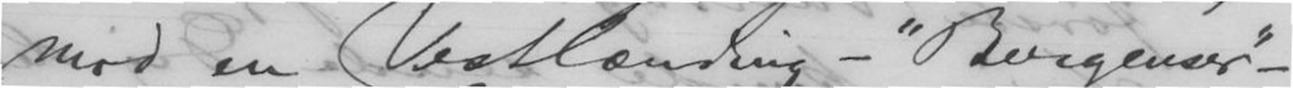mod en Vestlænding - "Bergenser" -
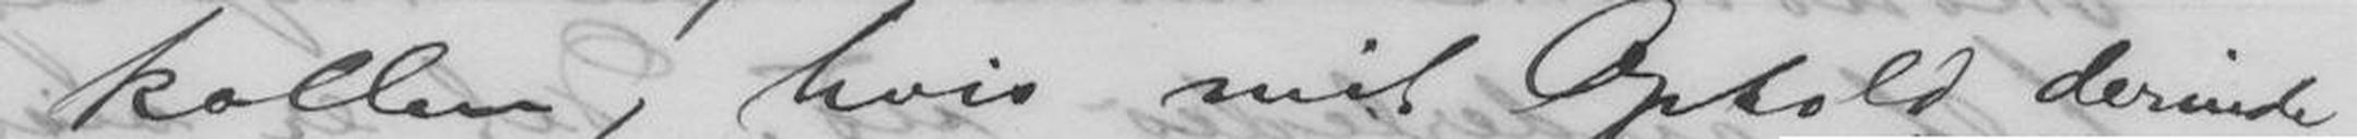kollen, hvis mit Ophold derinde
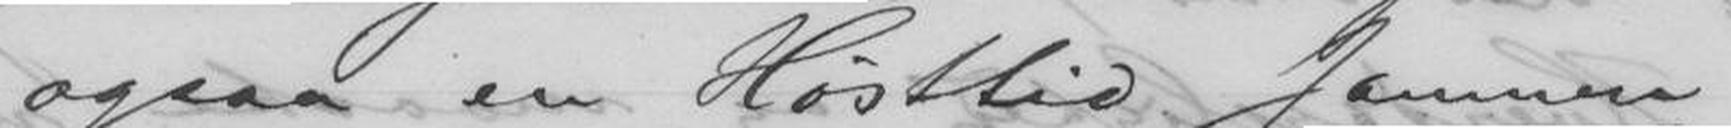ogsaa en Høsttid sammen, -
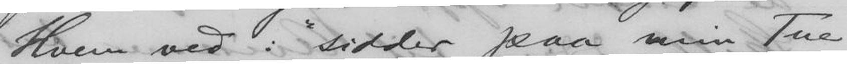Hvem ved: "sidder paa min Tue
